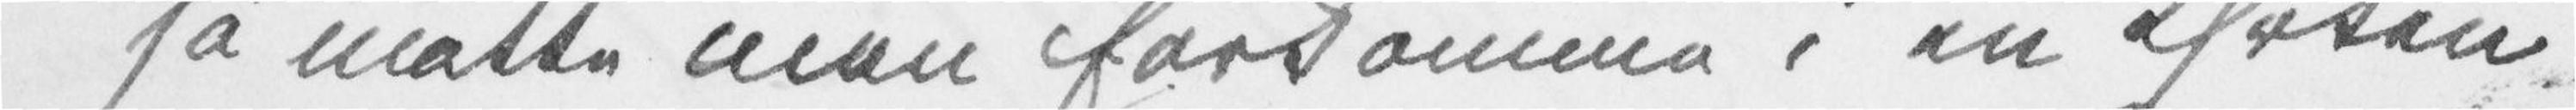så måtte man forkomme i en Ørken
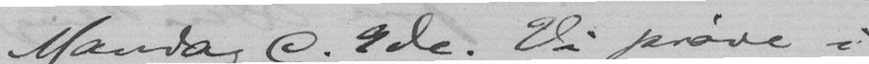Mandag d. 9de. Vi prøver i
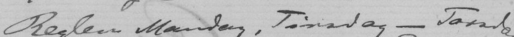Reglen Mandag, Tirsdag - Torsdag
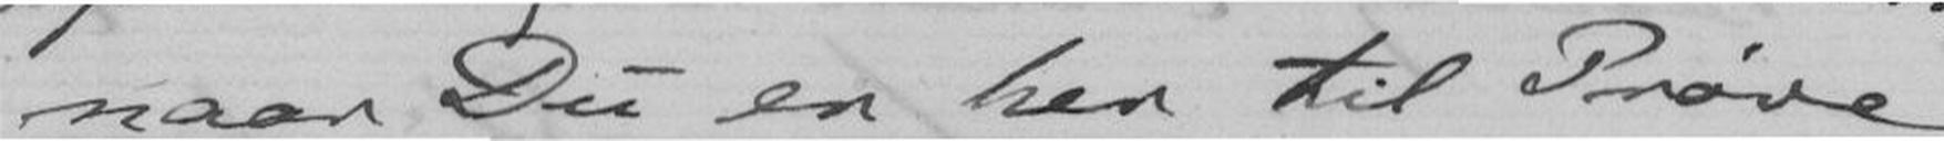naar Du er her til Prøve
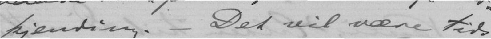kjending.- Det vil være tidsnok
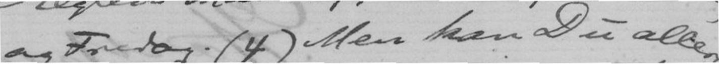og Fredag. (4) Men kan Du allerede
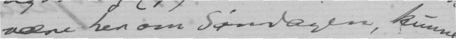være her om Søndagen, kunne
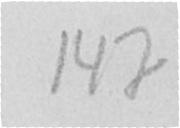147
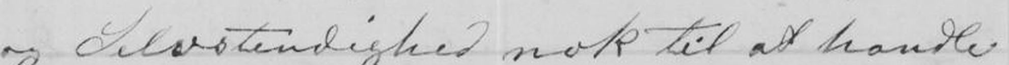og Selvstendighed nok til at handle
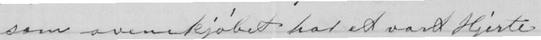som ovenikjøbet har et vart Hjerte
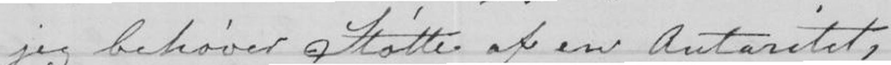jeg behøver støtte af en Autoritet,
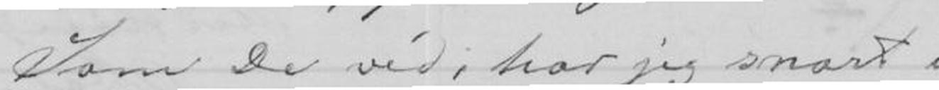Som du ved, har jeg snart i
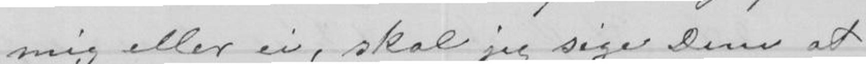mig eller ei, skal jeg sige Dem at
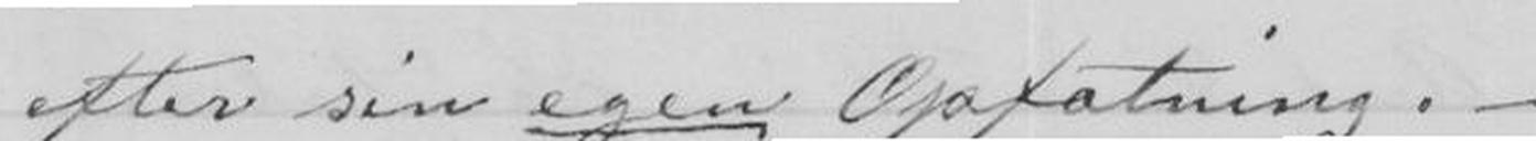efter sin egen Opfatning.-
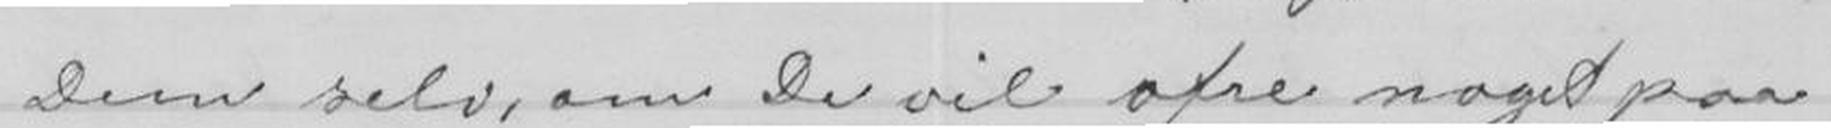Dem selv, om Du vil ofre noget paa
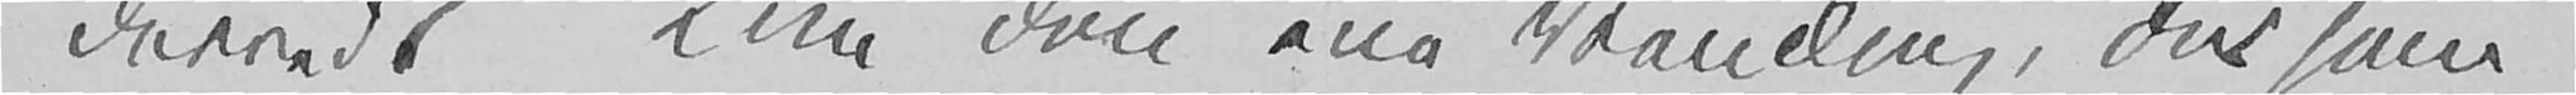derved. Kun den ene Vending, der som
